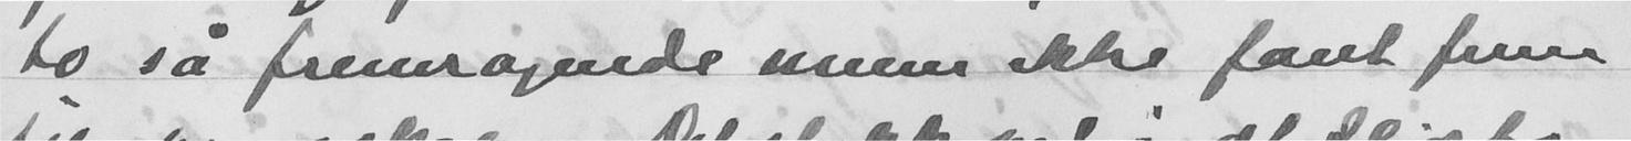to så fremragende menn ikke fant frem
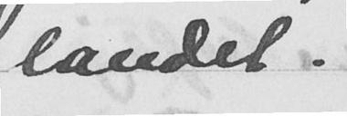landet.
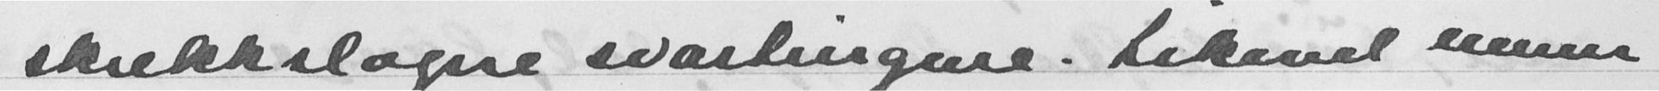skrekkslagne svartingene. Likevel mener
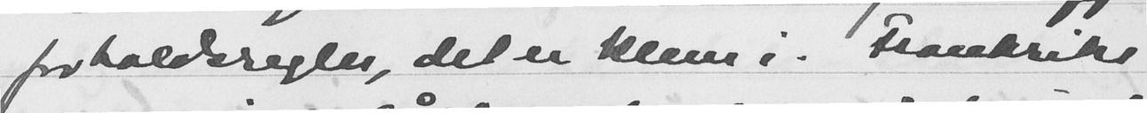forholdsregler, det er klem i. Frankrike
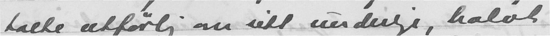talte utførlig om sitt underlige, halvt
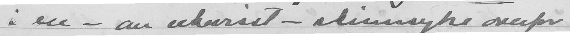i en - om ubevisst - skinnsyke overfor
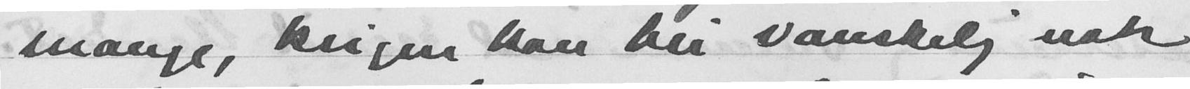mange, krigen kan bli vanskelig nok
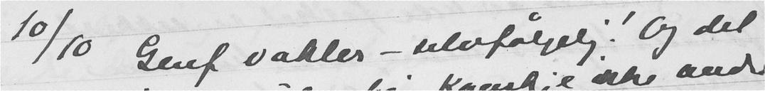10/10 Genf vakler - selvfølgelig! Og det
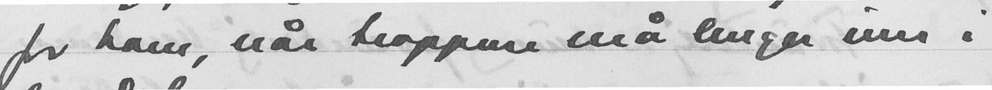for ham, når troppene må lenger nord i
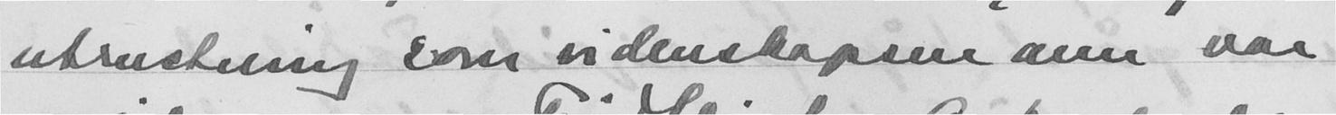utrustning som videnskapsmann var
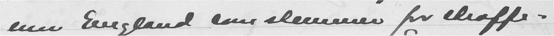enn England som stemmer for straffe-
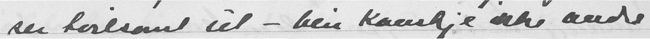ser tvilsomt ut - blir kanskje ikke andre
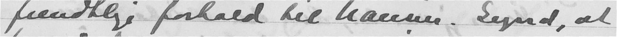fiendtlige forhold til Nansen. Synd, at
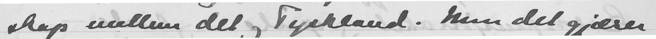skap mellom det og Tyskland. Men det gjærer
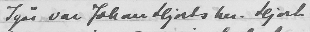Igår var Johan Hjort her. Hjort
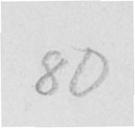80
